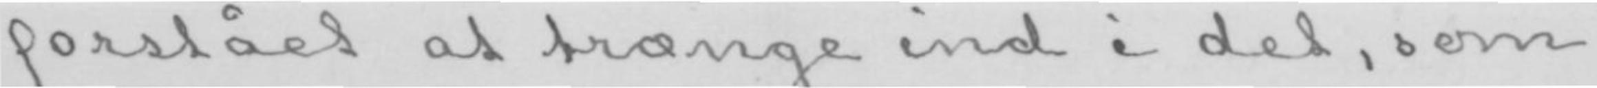forstået at trænge ind i det, som
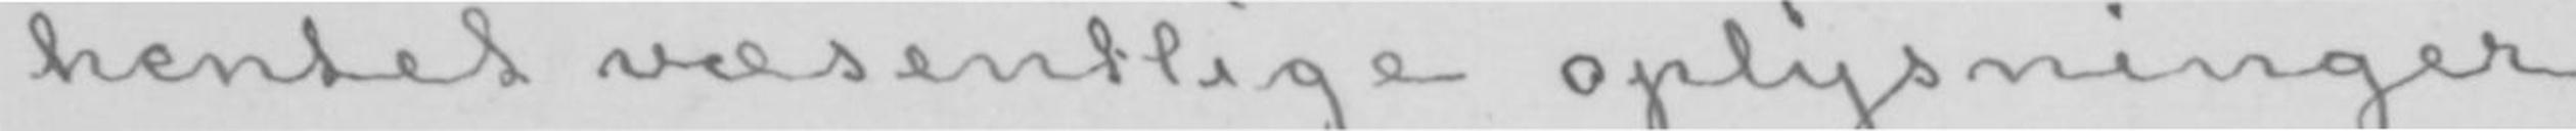hentet væsentlige oplysninger
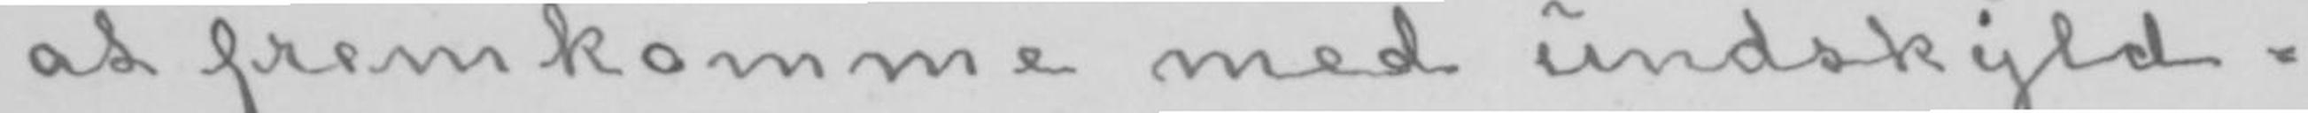at fremkomme med undskyld-
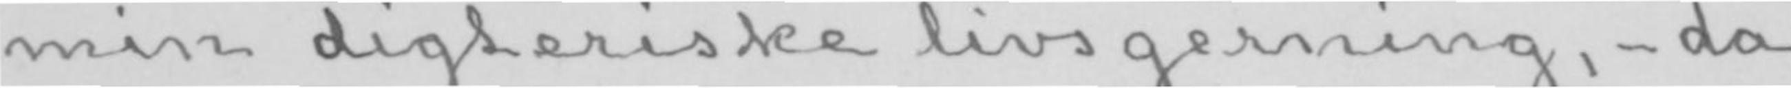min digteriske livsgerning,- da
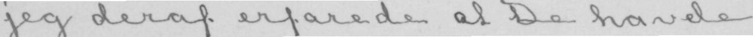jeg deraf erfarede at De havde
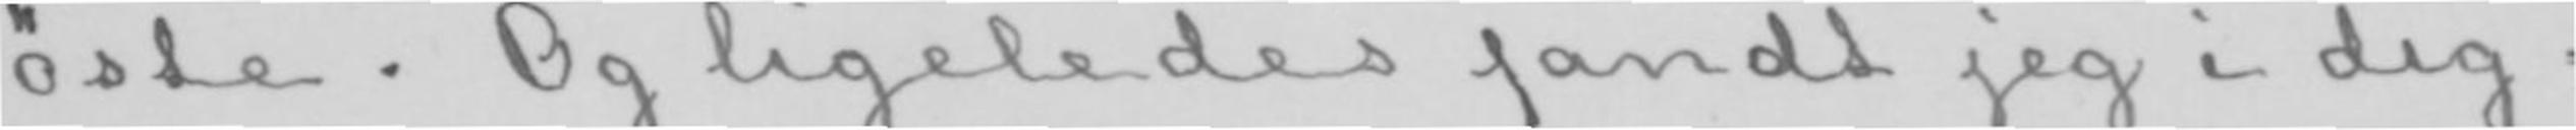øste. Og ligeledes fandt jeg i dig-
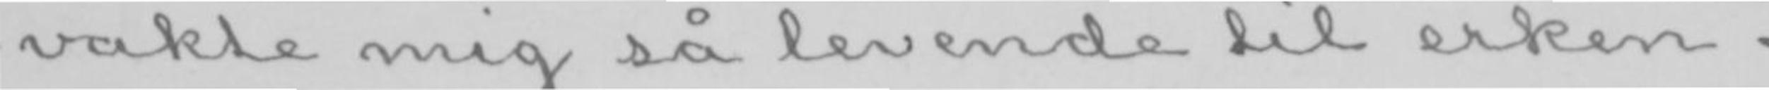vakte mig så levende til erken-
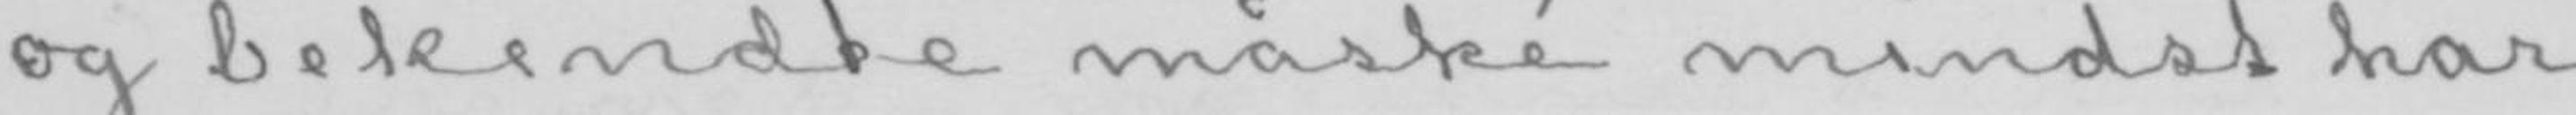og bekendte måské mindst har
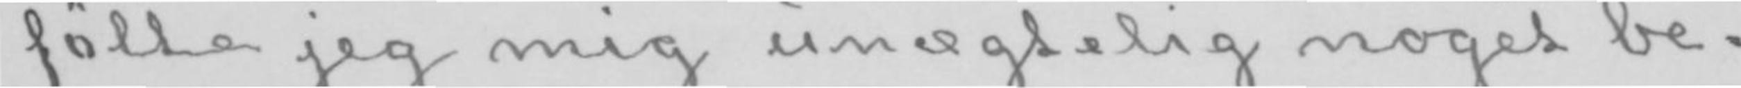følte jeg mig unægtelig noget be-
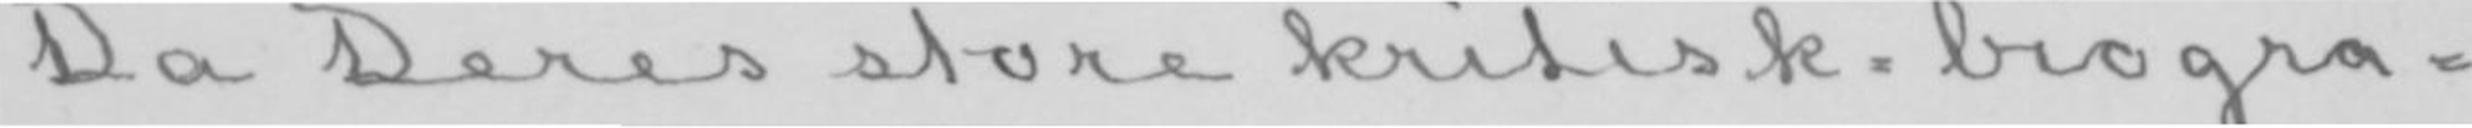Da Deres store kritisk-biogra-
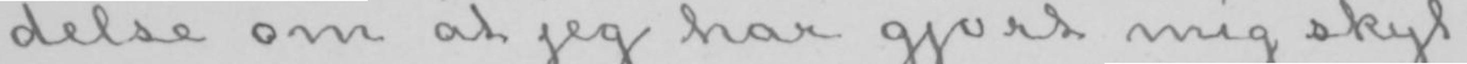delse om at jeg har gjort mig skyl-
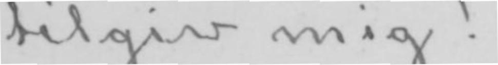tilgiv mig!
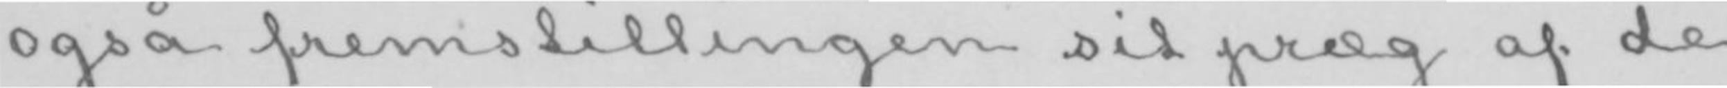også fremstillingen sit præg af de
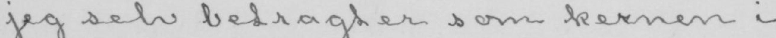jeg selv betragter som kernen i
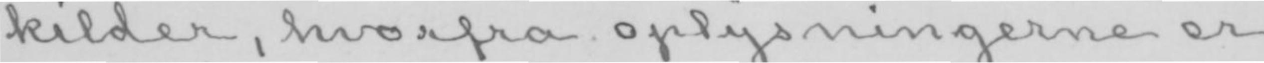kilder, hvorfra oplysningerne er
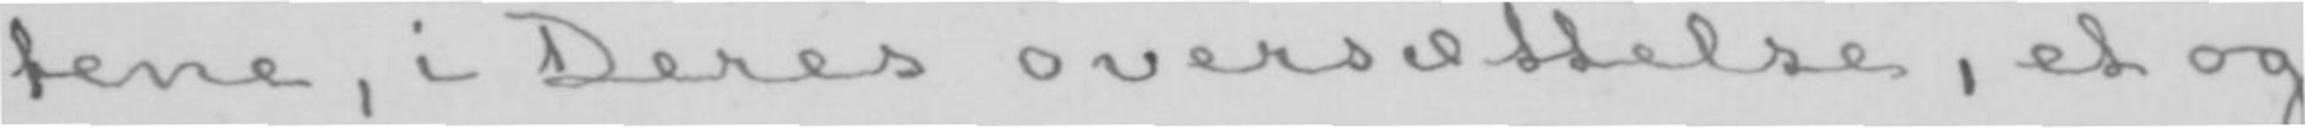tene, i Deres oversættelse, et og
